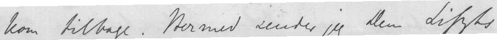kom tilbage. Hermed sender jeg Dem Liszts
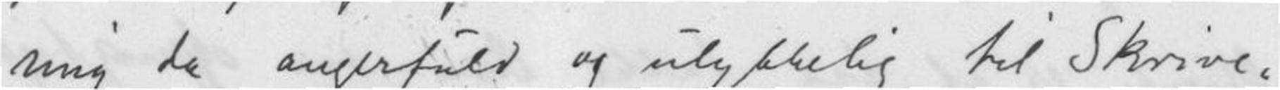mig da angerfuld og ulykkelig til Skrive-
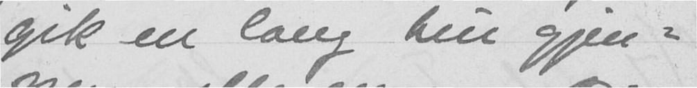gik en lang tur gjen-
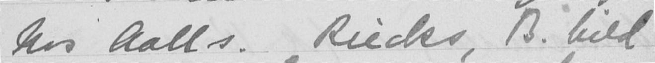hos Aalls. Riecks, B. hild
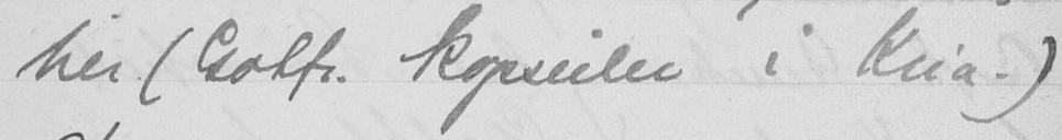her (Gotfr. kapseiler i Kria.)
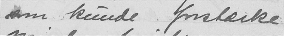som kunde forstærke
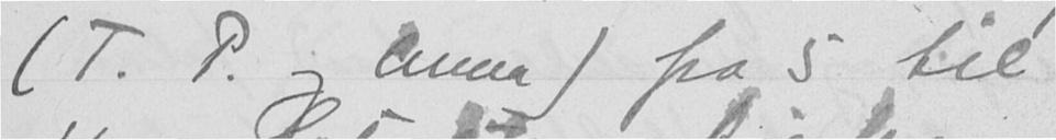(T. P. og Anna) fra 5 til
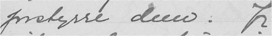forstyrre dem. Jeg
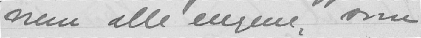men alle engene som
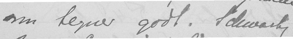som tegner godt. Schwartz
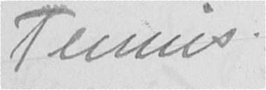Tennis.
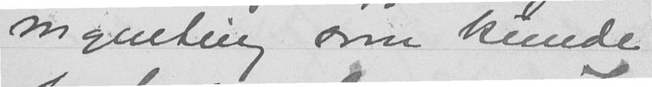ingenting som kunde
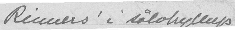Reimers i sølvbryllup
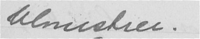blomstrer.
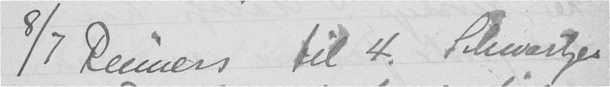8/7 Reimers til 4. Schwartzes
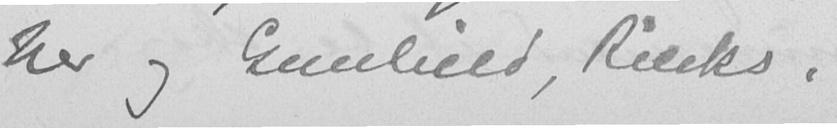her og Gunhild, Riecks.
